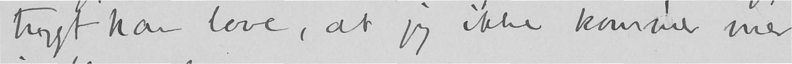trygt kan love, at jeg ikke kommer mer
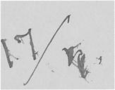17/4.
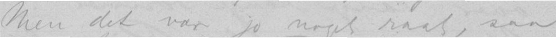Men det var jo noget raat, saa
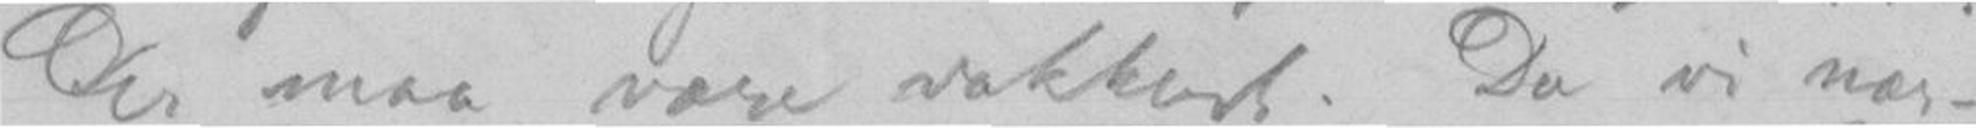Der maa være vakkert. Da vi mær-
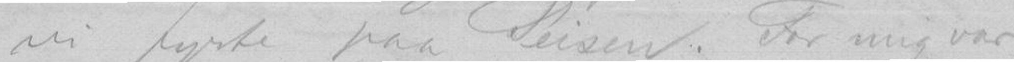vi fyrte paa Peisen. For mig var
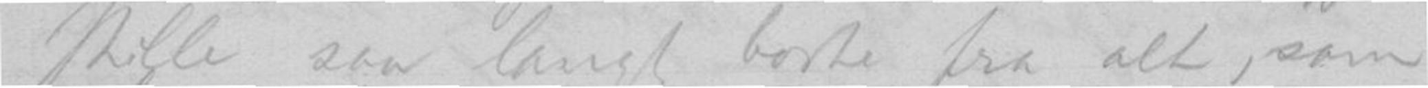stille saa langt borte fra alt, som
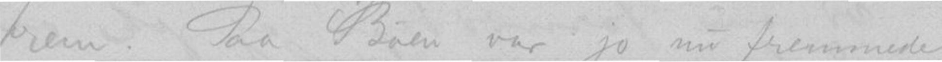frem. Paa Bøen var jo nu fremmede
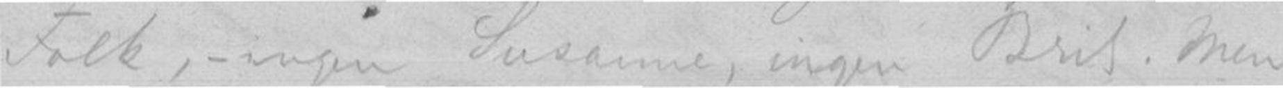Folk, - ingen Susanne, ingen Brit. Men
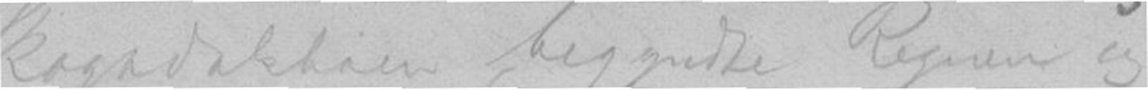os Skogadalsbøen begyndte Regnen og
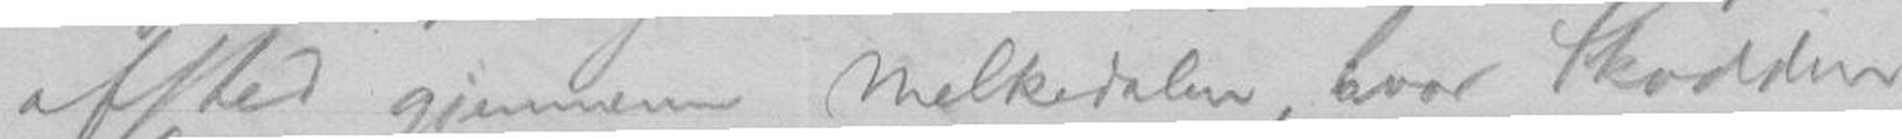afsted gjennem Melkedalen, hvor Skodden
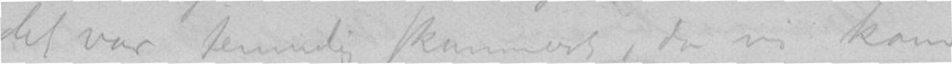det var temmelig skummert, da vi kom
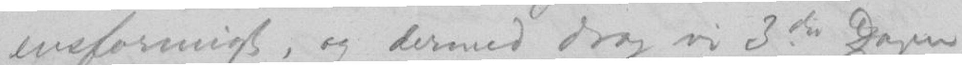ensformigt, og dermed drog vi 3die Dagen
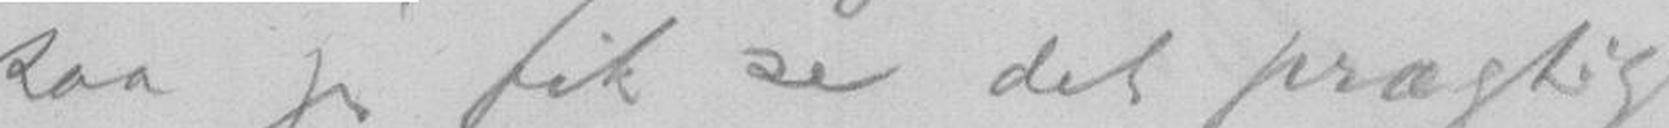saa jeg fik se det prægtige
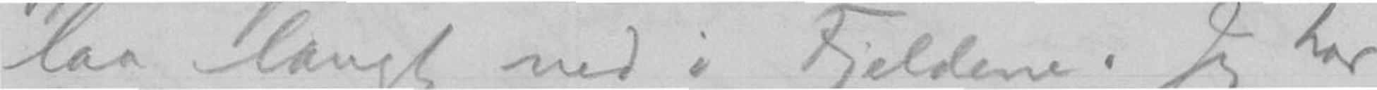laa langt ned i Fjeldene. Jeg har
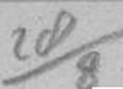28/83
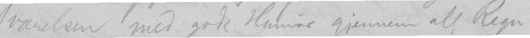værelsen med godt Humør gjennem alt Regn-
